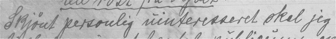Skjønt personlig uinteresseret skal jeg
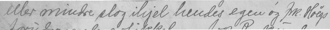eller mindre slog ihjel hendes egen og frk. Høegs
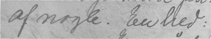af nogle. En hed:
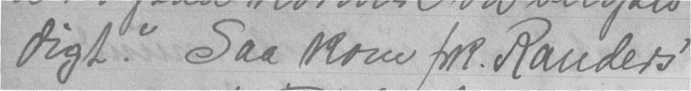digt." Saa kom frk. Randers'
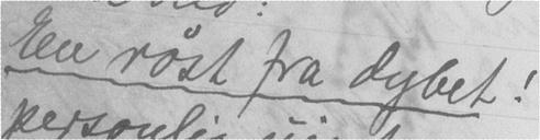En røst fra dybet!
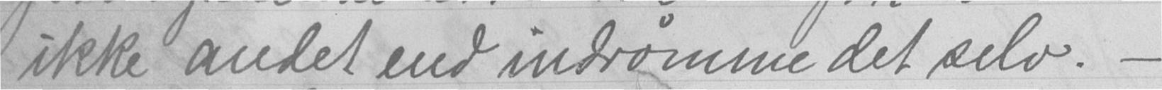ikke andet end indrømme det selv. -
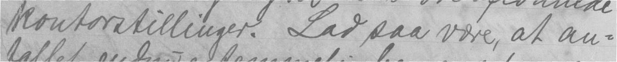kontorstillinger. Lad saa være, at an-
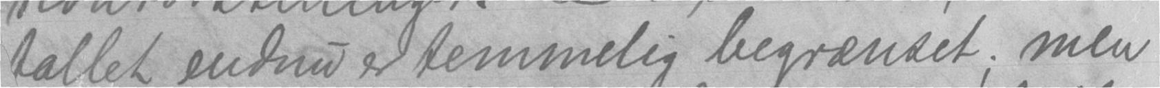tallet endnu er temmelig begrænset; men
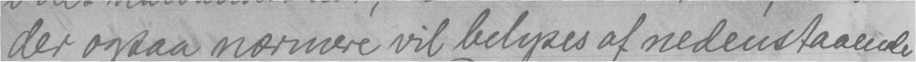der ogsaa nærmere vil belyses af nedenstaaende
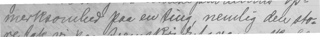merksomhed paa en ting, nemlig den sto-
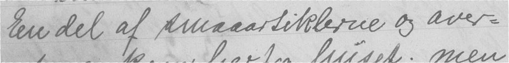En del af smaaartiklerne og aver-
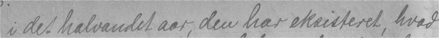i det halvandet aar, den har eksisteret, hvad
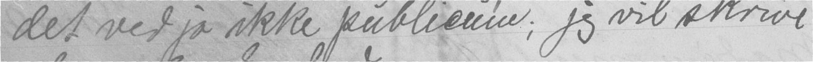det ved jo ikke publicum; jeg vil skrive
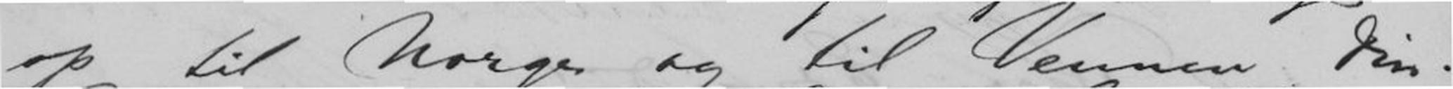op til Norge og til Vennen Din.
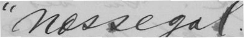"Næssegal".
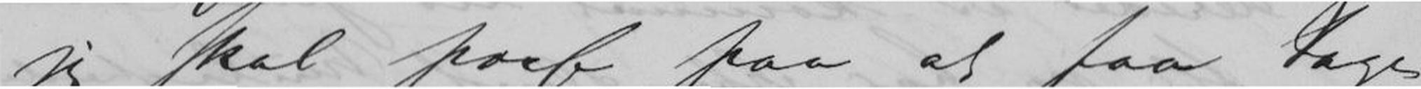jeg skal passe paa at faa tage
